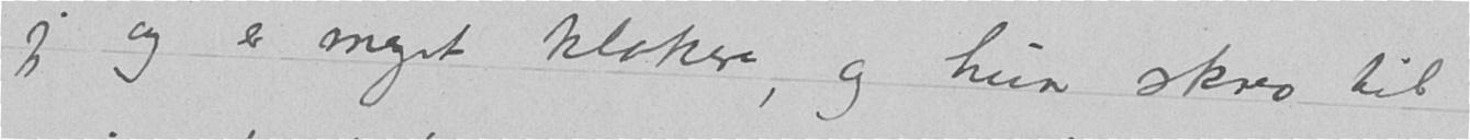jeg og er meget klokere, og hun skrev til
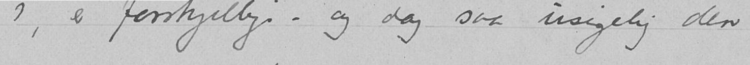i, er forskjellige - og dog saa usigelig den
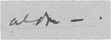aldre – .
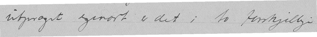utpræget egenart er det i to forskjellige
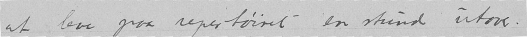at leve paa repertoiret - en stund utover.
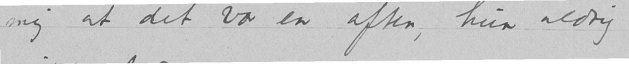mig at det var en aften, hun aldrig
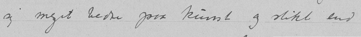seg meget bedre paa kunst og slikt end
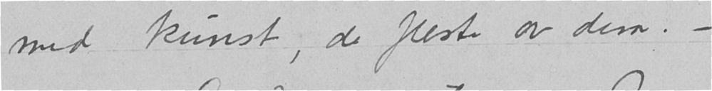med kunst, de fleste av dem. -
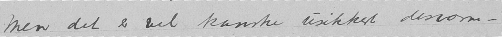Men det er vel kanske usikkert desværre -
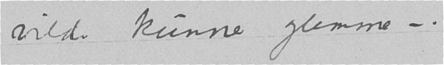vilde kunne glemme -.
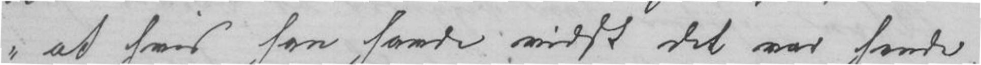at hvis han havde vidst det var hende,
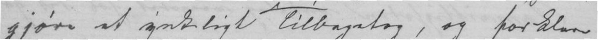gjøre et ynkeligt tilbagetog, og forslaar
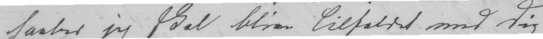haaber jeg selv bliver tilfældet med Dig,
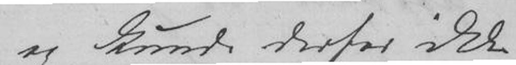og kunde derfor ikke
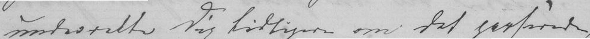underrette Dig tidligere om det indtrufne,
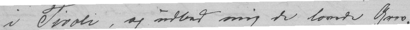i Tivoli, og udbad mig de lovede Grov-
